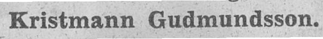Kristmann Gudmundsson.
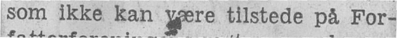som ikke kan være tilstede på For-
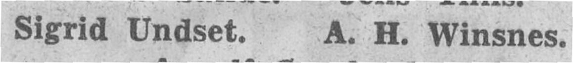Sigrid Undset. A. H. Winsnes.
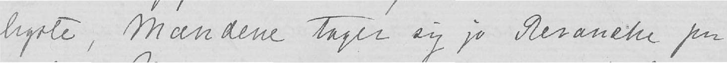ligste, Mændene tager sig jo Revanche paa
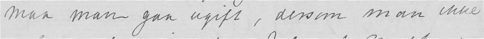maa man gaa ugift, dersom man ikke
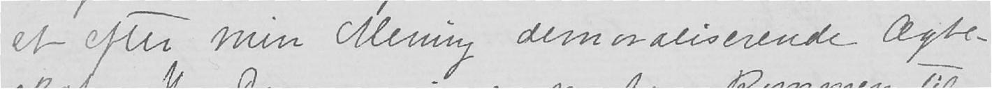et efter min Mening demoraliserende Ægte-
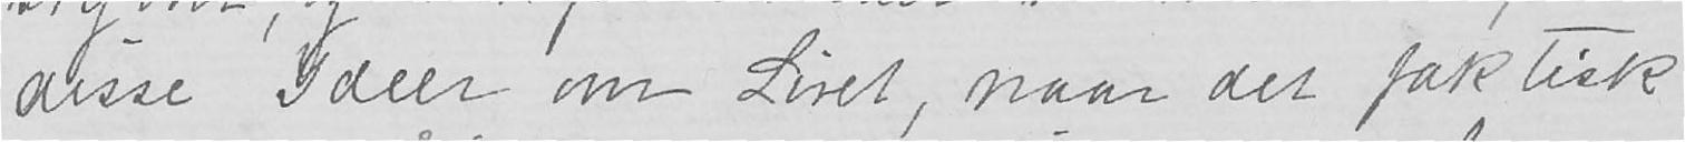disse Ideer om Livet, naar det faktisk
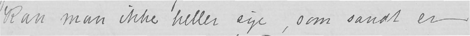Kan man ikke heller sige, som sandt er, –
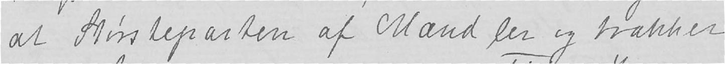at Størsteparten af Mænd ler og trækker
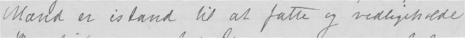Mænd er istand til at fatte og vedligeholde
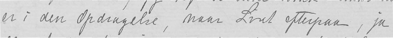er i den Opdragelse, naar Livet efterpaa, ja
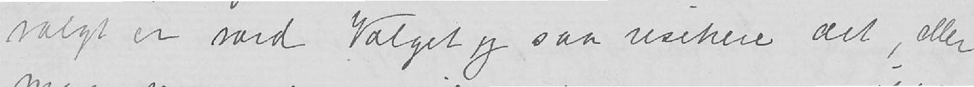valgt er værd Valget og saa resikere det, eller
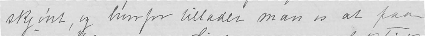skjønt, og hvorfor tillader man os at faa
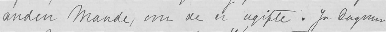anden Maade, om de er ugifte. Ja Dagmar
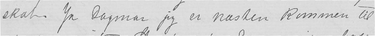skab. Ja Dagmar jeg er næsten kommen til
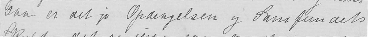saa er det jo Opdragelsen og Samfundets
